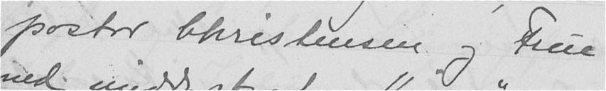pastor Christensen og Frue
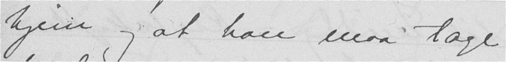hjem og at han maa tage
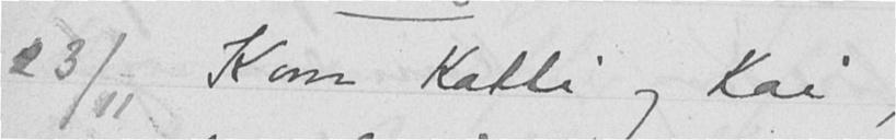23/11 Kom Katti og Kai,
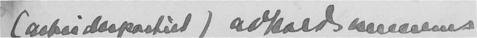(arbeiderpartiet) avholdt ord
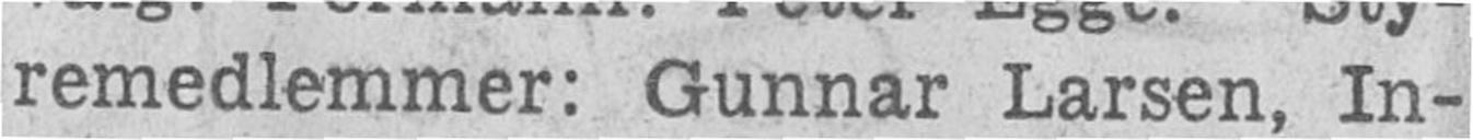remedlemmer: Gunnar Larsen, In-
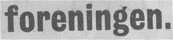foreningen
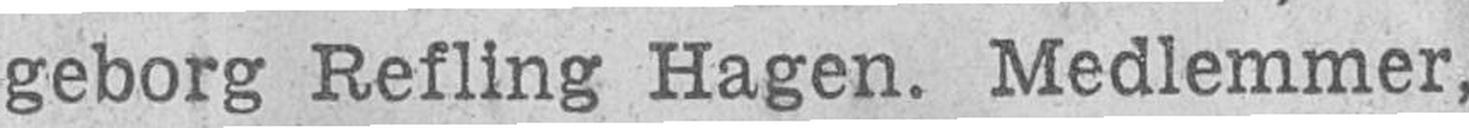geborg Refling Hagen. Medlemmer,
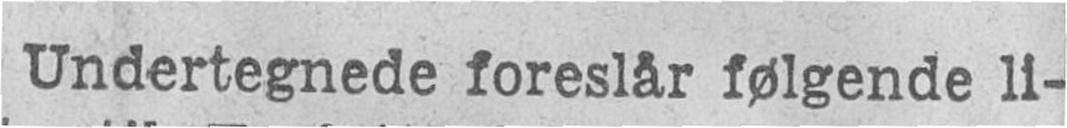Undertegnede foreslår følgende li-
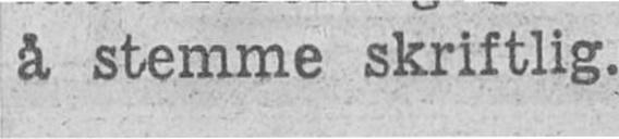å stemme skriftlig.
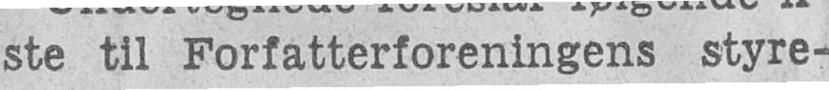ste til Forfatterforeningens styre-
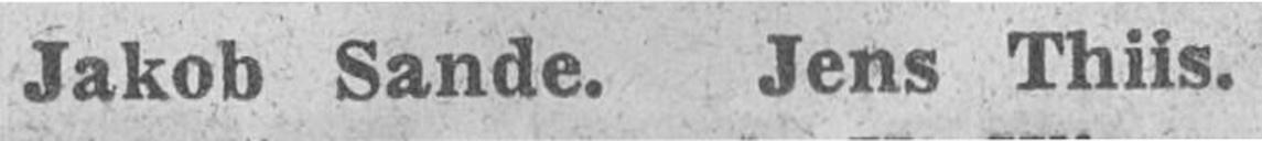Jakob Sande. Jens Thiis.
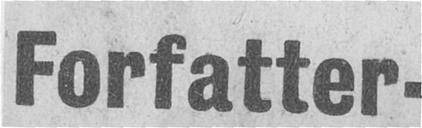Forfatter-
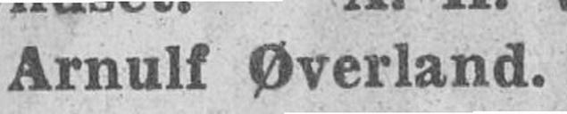Arnulf Øverland.
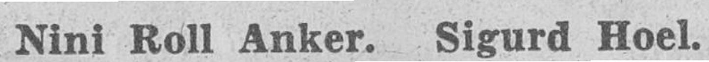Nini Roll Anker. Sigurd Hoel.
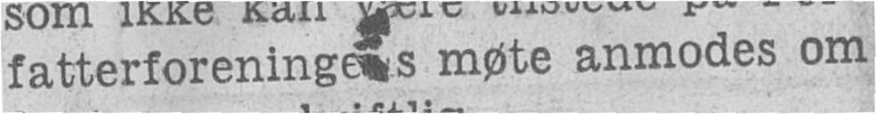fatterforeningens møte anmodes om
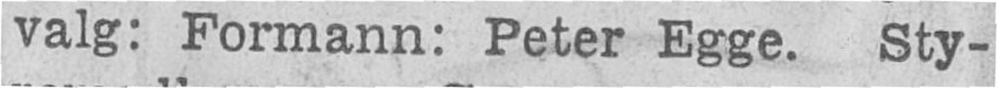valg: Formann: Peter Egge. Sty-
